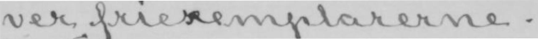ver friexemplarerne.
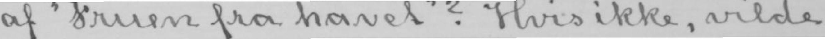af «Fruen fra havet»? Hvis ikke, vilde
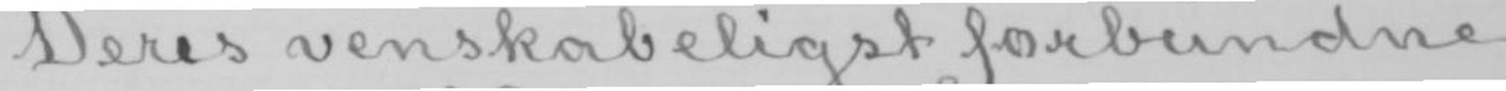Deres venskabeligst forbundne
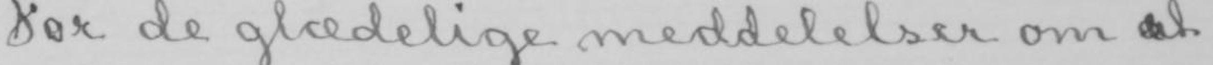For de glædelige meddelelser om aet
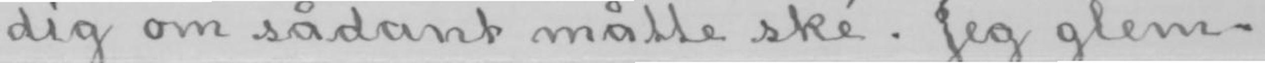dig om sådant måtte ské. Jeg glem-
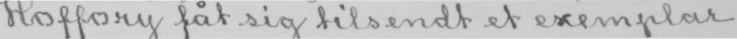Hoffory fåt sig tilsendt et exemplar
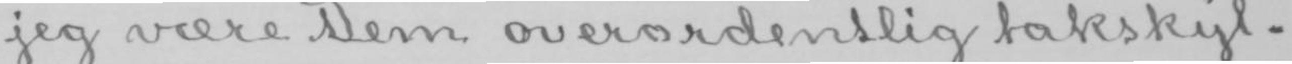jeg være Dem overordentlig takskyl-
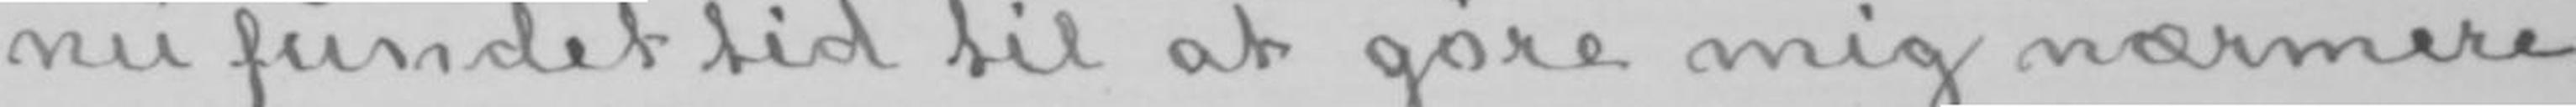nu fundet tid til at gøre mig nærmere
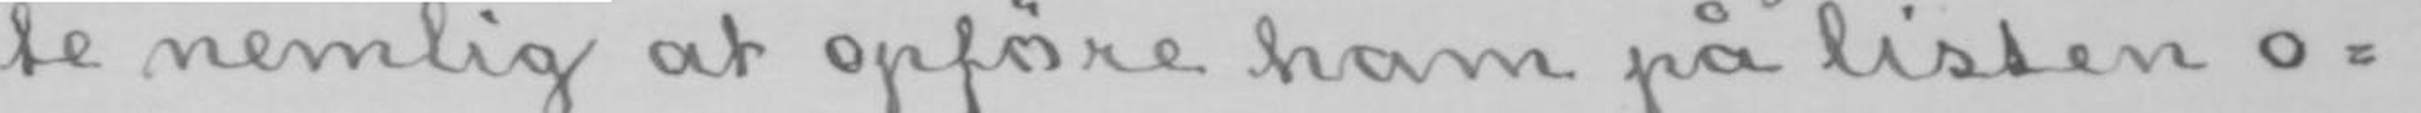te nemlig at opføre ham på listen o-
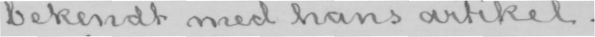bekendt med hans artikel.
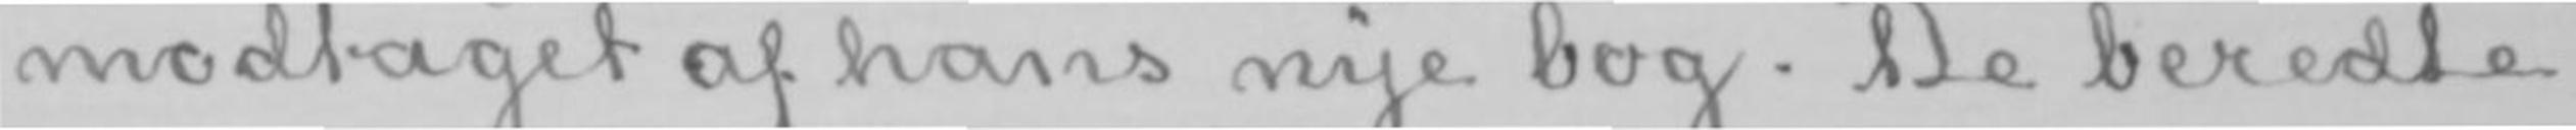modtaget af hans nye bog. De beredte
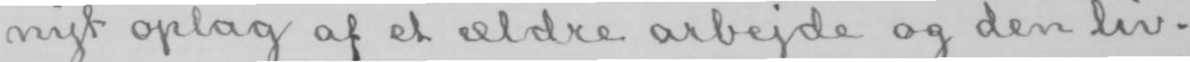nyt oplag af et ældre arbejde og den liv-
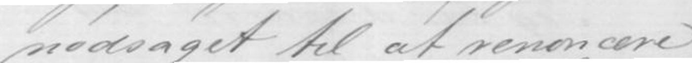nødsaget til at renoncere
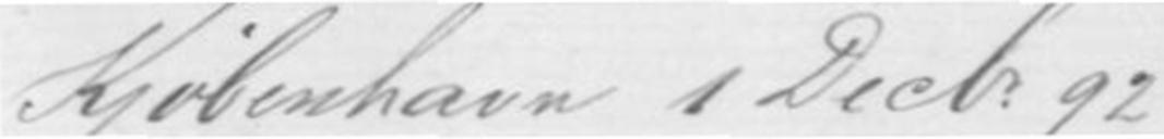Kjøbenhavn 1 Decbr. 92
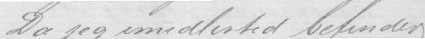Da jeg imidlertid befinder
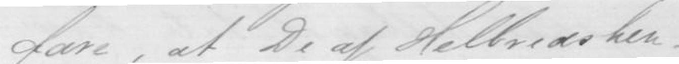fare, at De af Helbredshen-
